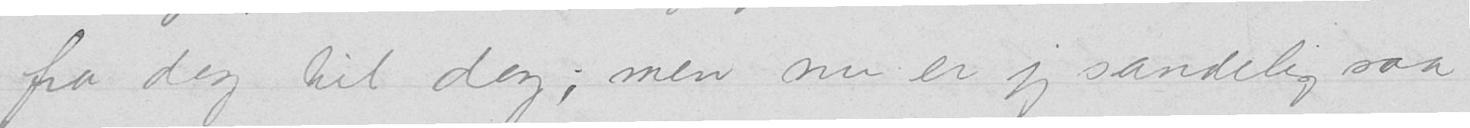fra dag til dag; men nu er jeg sandelig saa
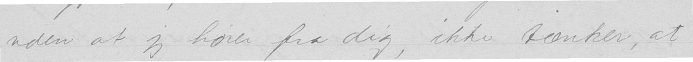uden at jeg hører fra dig, ikke tænker, at
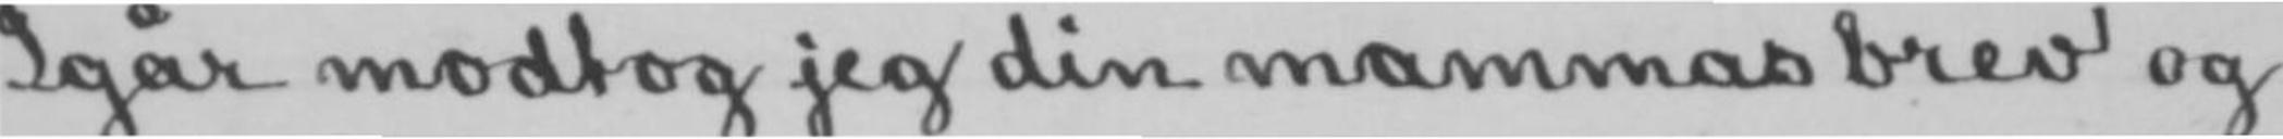Igår modtog jeg din mammas brev og
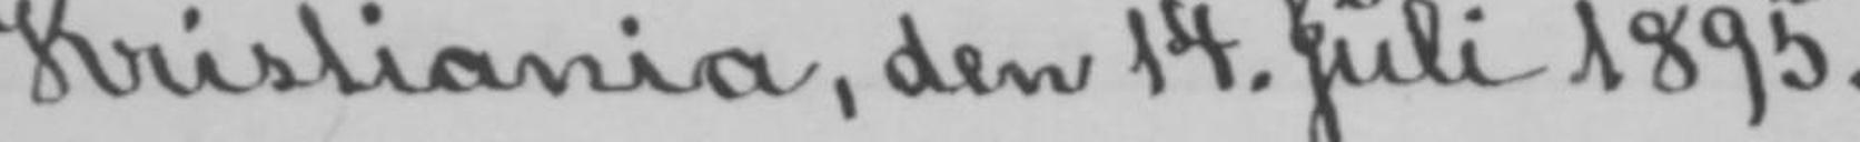Kristiania, den 14. Juli 1895.
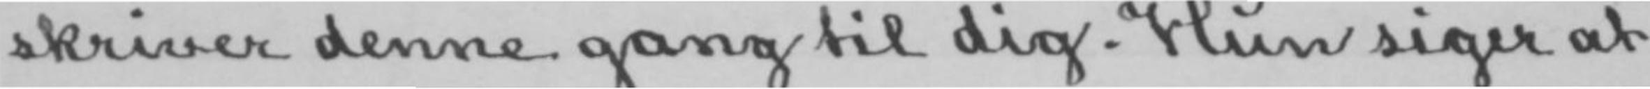skriver denne gang til dig. Hun siger at
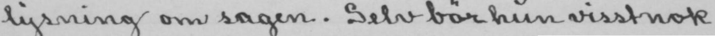lysning om sagen. Selv bør hun visstnok
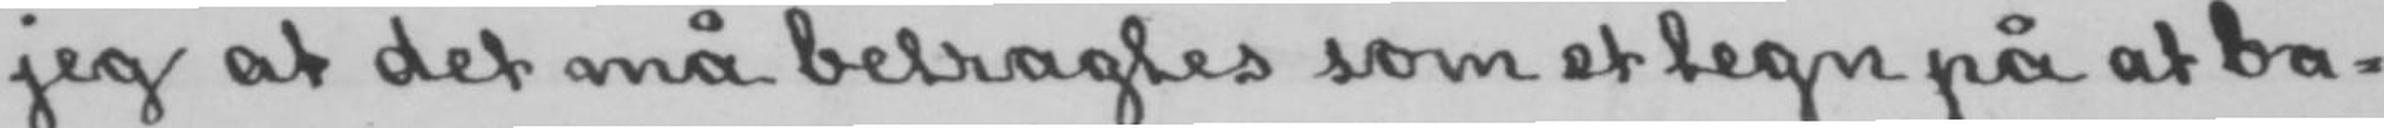jeg at det må betragtes som et tegn på at ba-
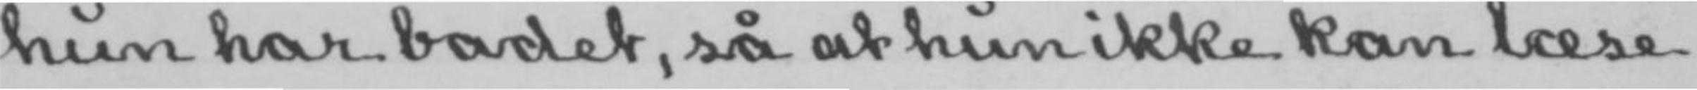hun har badet, så at hun ikke kan læse
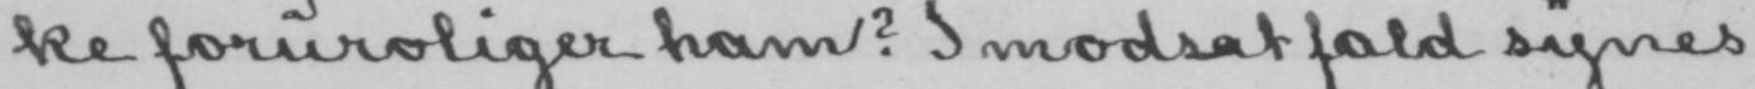ke foruroliger ham? I modsat fald synes
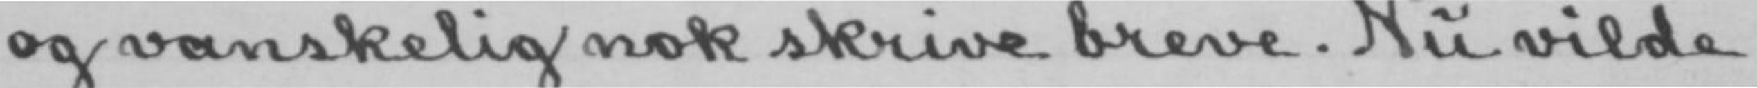og vanskelig nok skrive breve. Nu vilde
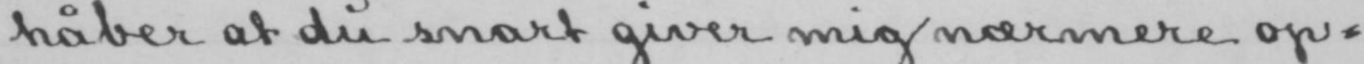håber at du snart giver mig nærmere op-
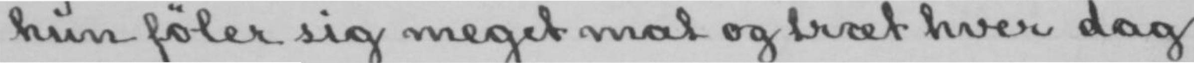hun føler sig meget mat og træt hver dag
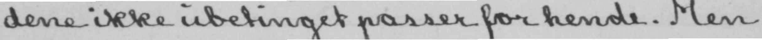dene ikke ubetinget passer for hende. Men
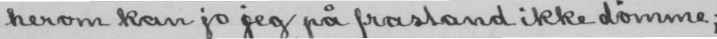herom kan jo jeg på frastand ikke dømme;
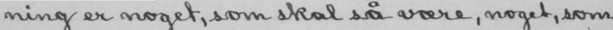ning er noget, som skal så være, noget, som
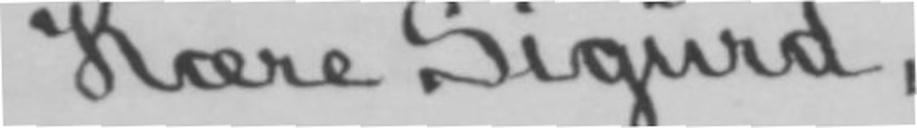Kære Sigurd,
